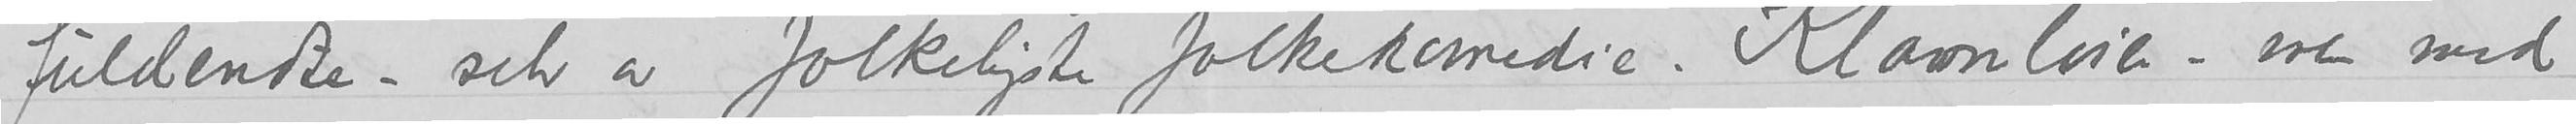fuldendte – selv av folkeligste folkekomedie. Klovnløier men med
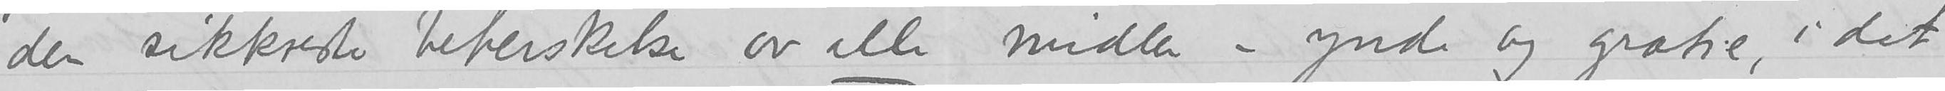den sikkreste beherskelse av alle midler – ynde og gratie, i det
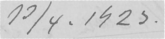12/4-1923.
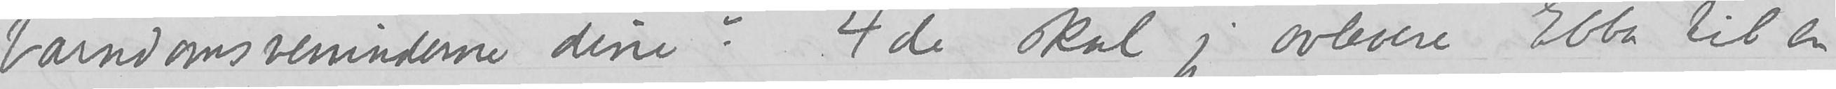barndomsminderne dine? 4de skal jeg overlevere Ebba til en
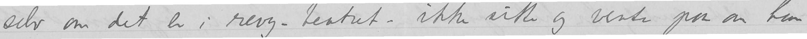selv om det er i revy-teatret – ikke sitte og vente paa om han
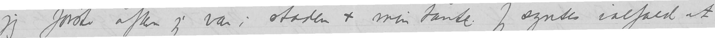jeg første aften jeg var i staden + min tante. Jeg syntes ialfald at
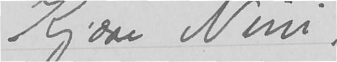Kjære Nini,
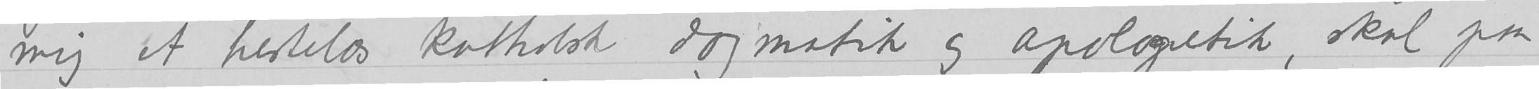mig et  hestelas  katholsk dogmatik og apologetik, skal paa
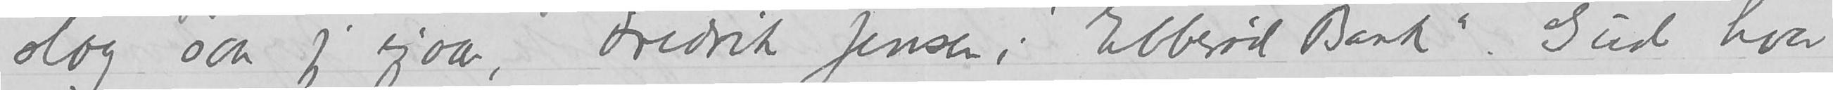slag saa jeg igaard, Fredrik Jensen i «Ebberød Bank». Gud hvor
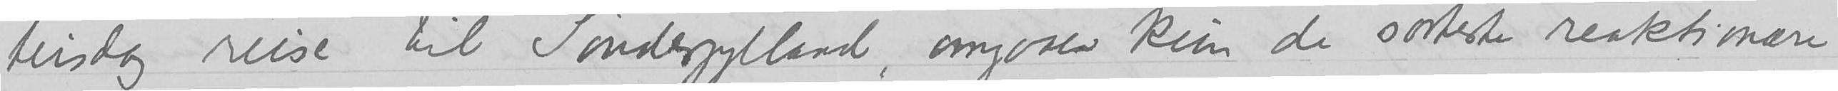tirsdag reise til Sønderjylland, omgaaes kun de sorteste reaktionære
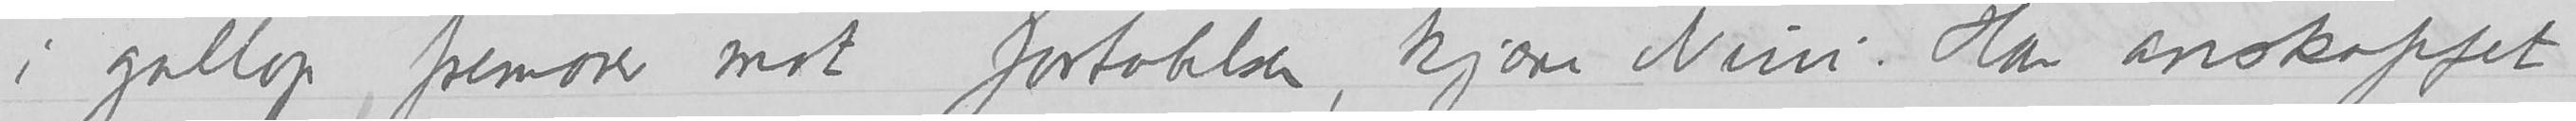i gallop fremover mot fortabelser, kjære Nini. Har anskaffet
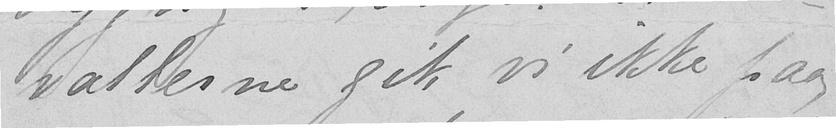vallerne gik vi ikke paa,
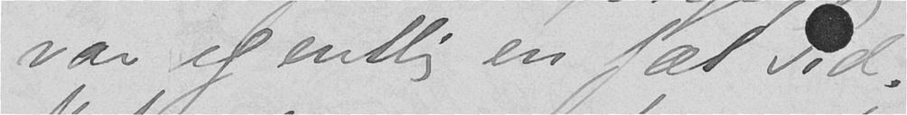var egentlig en fæl Tid.
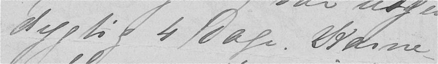dygtig 4 Dage. Karne-
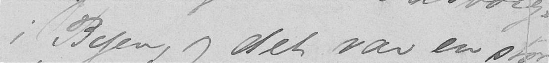i Byen, og det var en stor
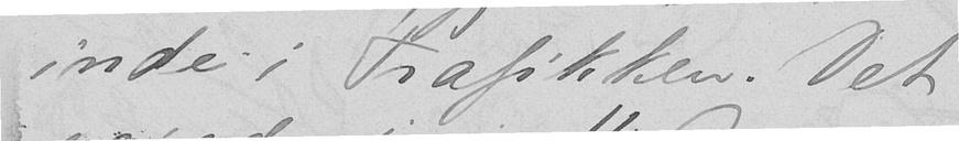inde i Trafikken. Det
